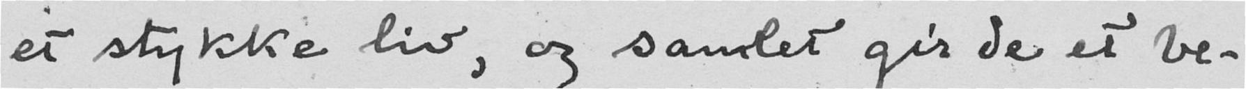et stykke liv, og samlet gir de et be-
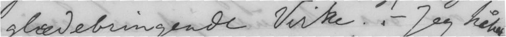glædebringende Virke.! - Jeg håber,
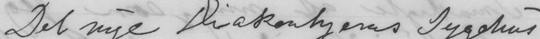Det nye Diakonhjems Sygehus
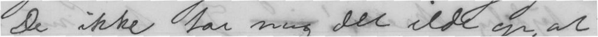De ikke tar mig det ilde op, at
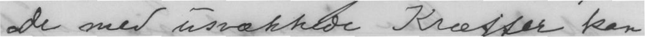De med usvækkede Kræfter kan
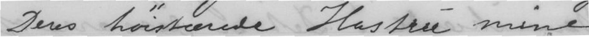Deres høistærede Hustru mine
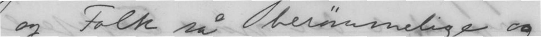og Folk så berømmelige og
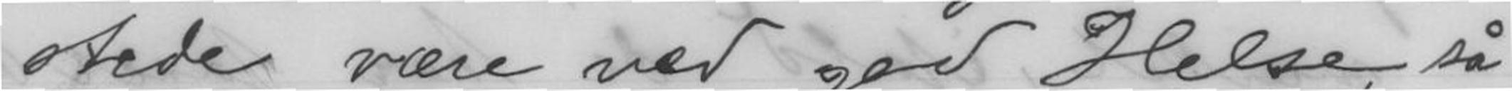stede være ved god Helse, så
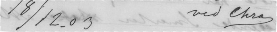18/12.03 ved Chra
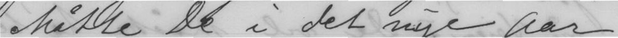Måtte De i det nye Aar
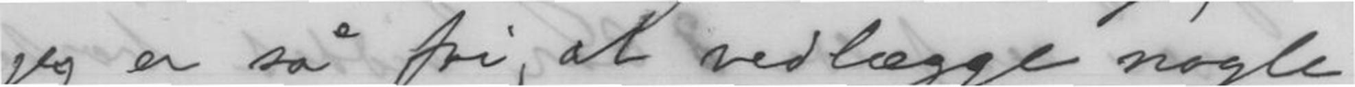jeg er så fri, at vedlægge nogle
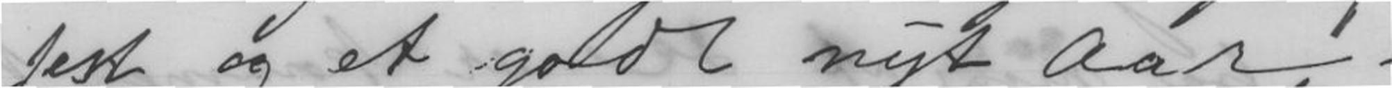fest og et godt nyt Aar!
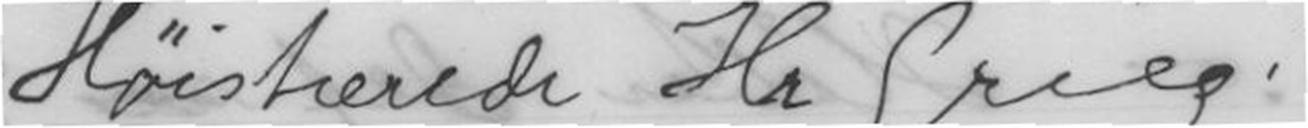Høistærede Hr Grieg!
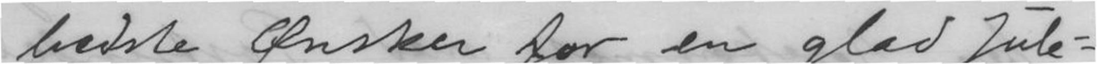bedste Ønsker for en glad Jule-
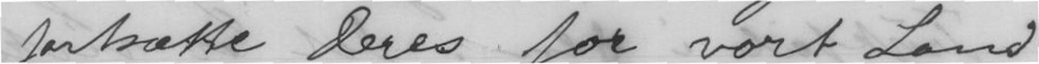fortsætte Deres for vort Land
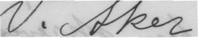V. Aker
